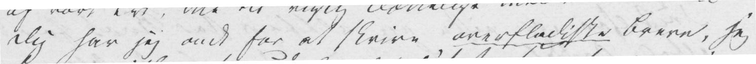Dig har jeg ondt for at skrive overfladiske Breve, jeg
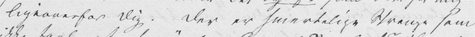ligeoverfor Dig. Der er smertelige Strenge som
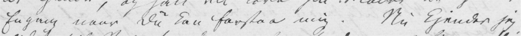Engang naar Du kan forstaa mig. Nu kjender jeg
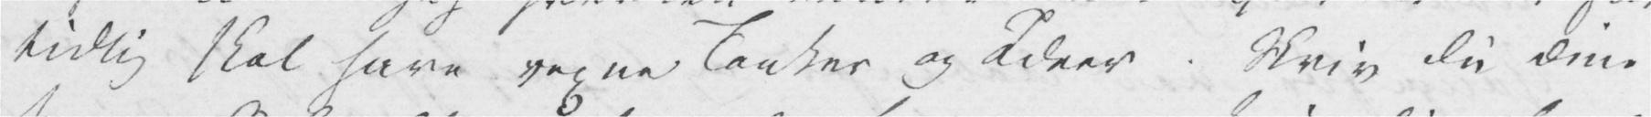tidlig skal have voxne Tanker og Ideer. Skriv Du Dine
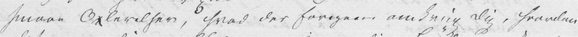smaae Oplevelser, hvad der foregaaer omkring Dig, hvordan
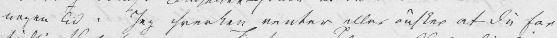nogen Tid. Jeg hverken venter eller ønsker at Du for
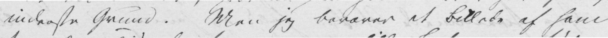inderste Grund. Men jeg bevarer et Billede af ham
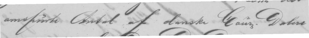omspurte Antal af danske Cours. Dalere
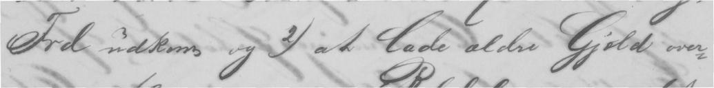Frd udkom og 2) at lade ældre Gjæld over-
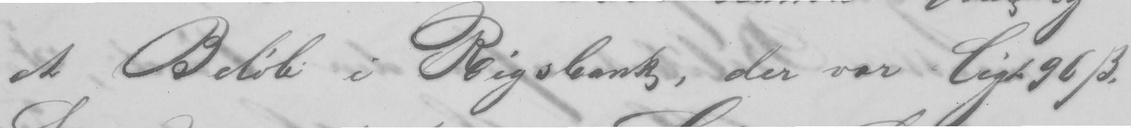et Beløb i Rigsbank, der var ligt 96 ẞ.
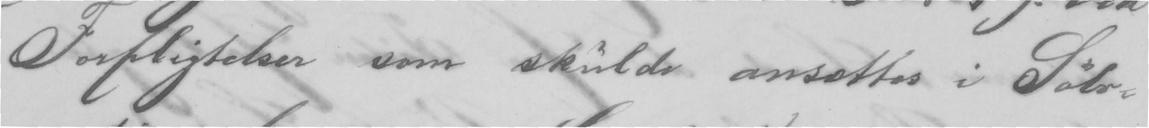Forpligtelser som skulde omsættes i Sølv-
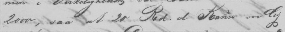2000, saa at 20 Rd. d. Kurs var lig
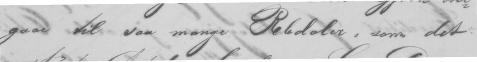gaae til saa mange Rbdaler, som det
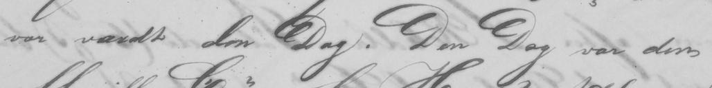var værdt den Dag. Den Dag var dens
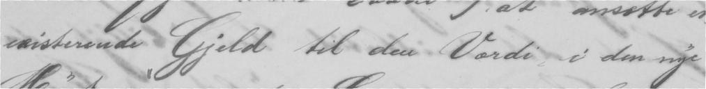existerende Gjeld til den Værdi i den nye
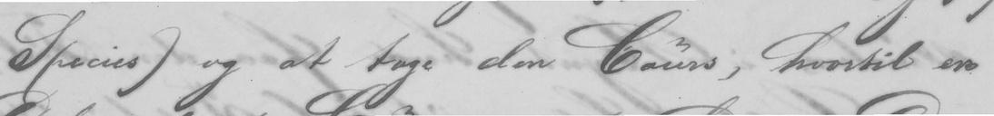Species) og at tage den Cours, hvortil en
